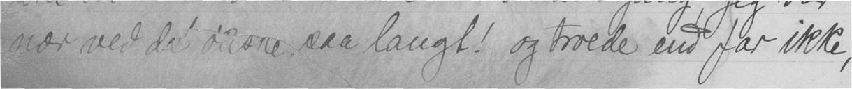nær ved d  saa langt! og troede end far ikke,
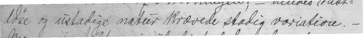løse og ustadige natur krævede stadig variation. -
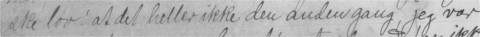ske lov! at det heller ikke den anden gang, jeg var
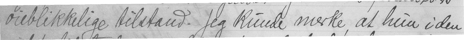øieblikkelige tilstand. Jeg kunde merke, at hun i den
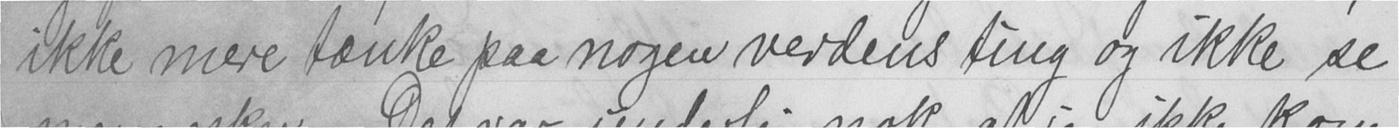ikke mere tænke paa nogen verdens ting og ikke se
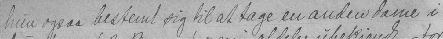hun ogsaa bestemt sig til at tage en anden dame i
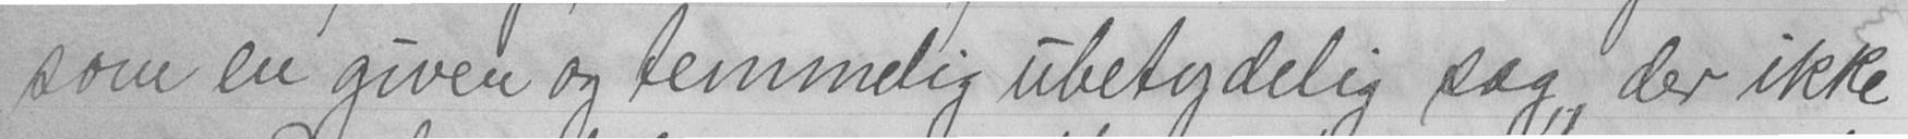som en given og temmelig ubetydelig sag, der ikke
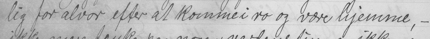lig for alvor efter at komme i ro og være hjemme, -
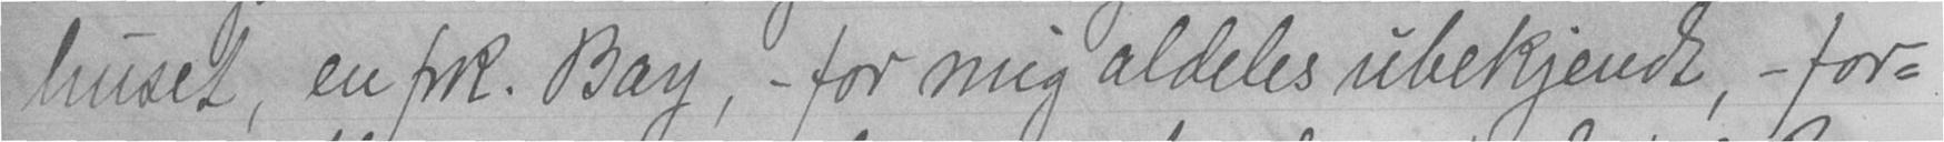huset, en frk. Bay, - for mig aldeles ubekjendt, - for-
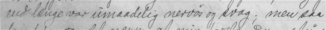end længe var umaadelig nervøs og svag; men saa
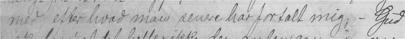med, efter hvad man senere har fortalt mig; - Gud
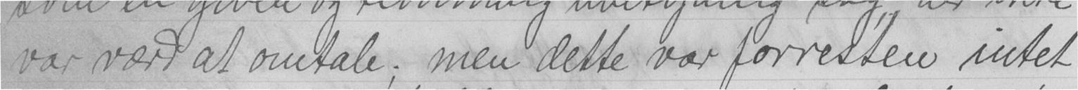var værd at omtale; men dette var forresten intet
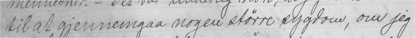til at gjennemgaa nogen større sygdom, om jeg
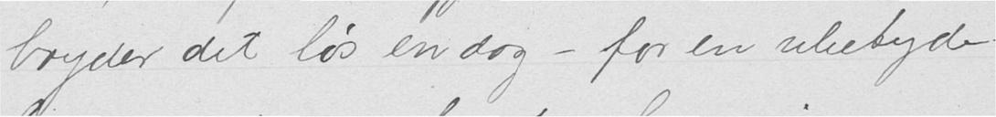bryder det løs en dag – for en ubetyde-
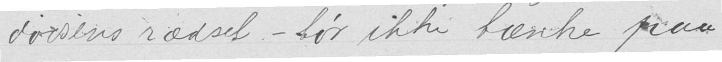dødsens rædsel – tør ikke tænke paa
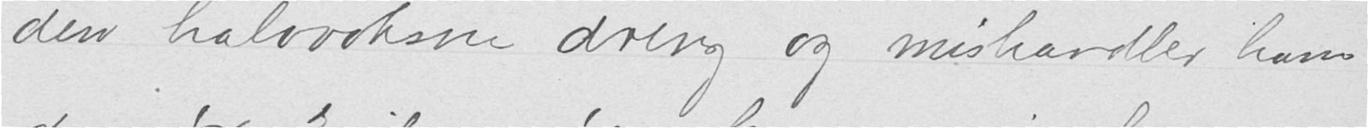den halvvoksne dreng og mishandler ham
